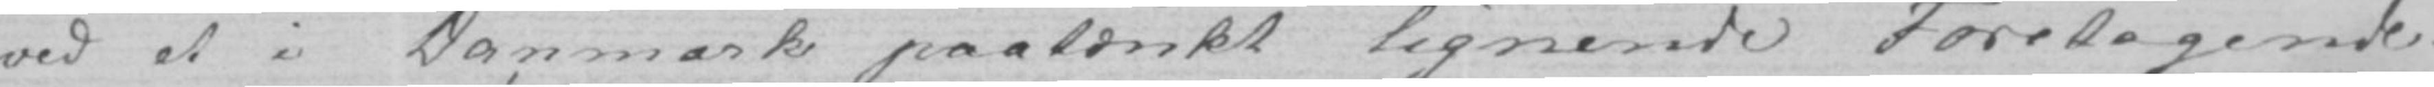ved et i Danmark paatænkt lignende Foretagende.
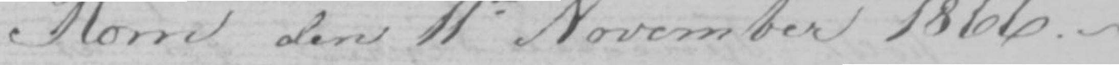Rom den 11te November 1866.-
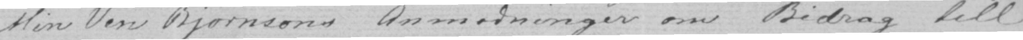Min Ven Bjørnsons Anmodninger om Bidrag till
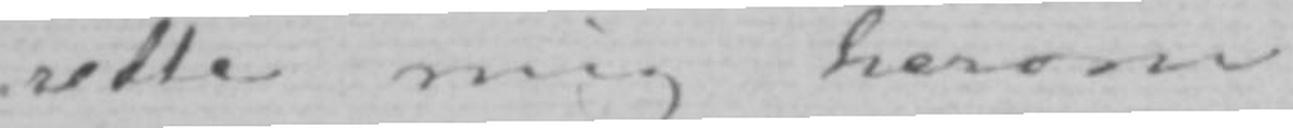rette mig herom.
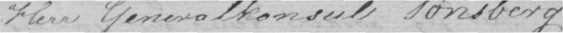Herr Generalkonsul Tønsberg!
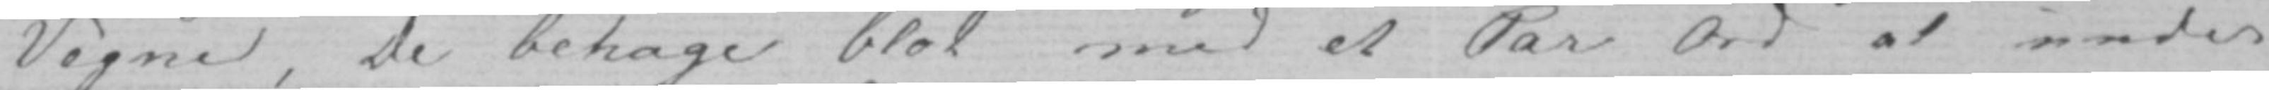Vegne, De behage blot med et Par Ord at under-
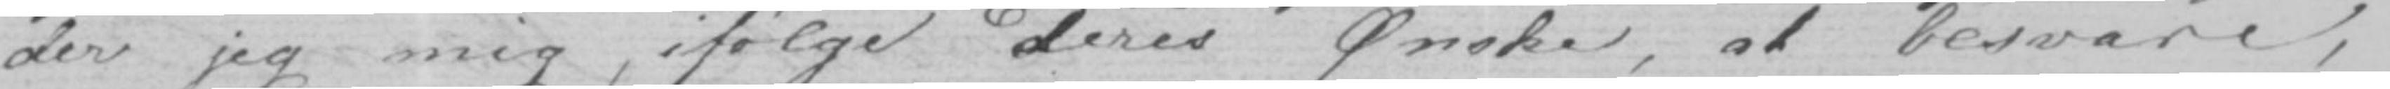der jeg mig, ifølge Deresderes Ønske, at besvare,
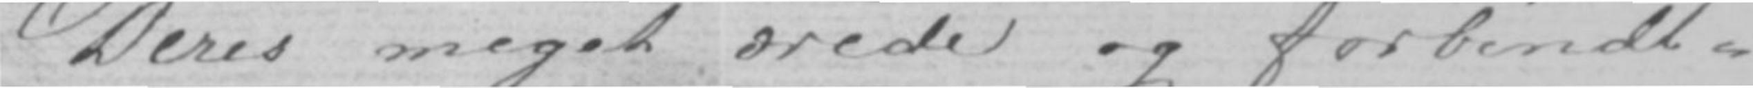Deres meget ærede og forbindt-
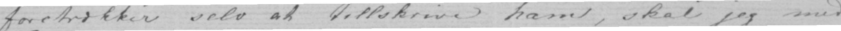foretrækker selv at tillskrive ham, skal jeg med
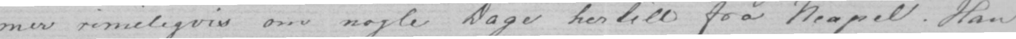mer rimeligvis om nogle Dage hertill fra Neapel. Han
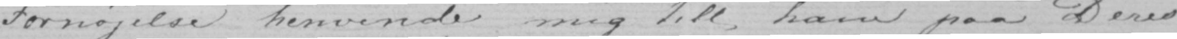Fornøjelse henvende mig till ham paa Deres
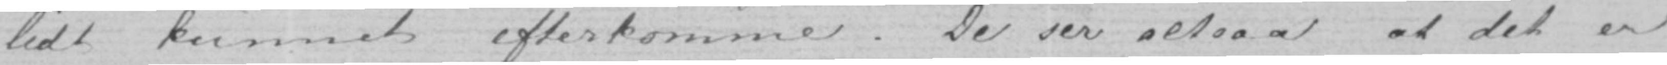lidt kunnet efterkomme. De ser altsaa at det er
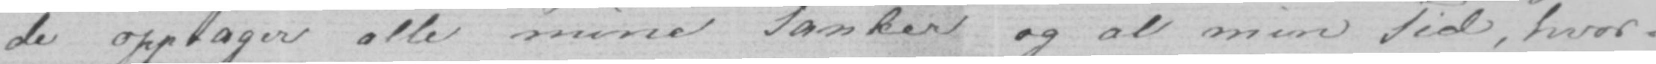de opptager alle mine Tanker og al min Tid, hvor-
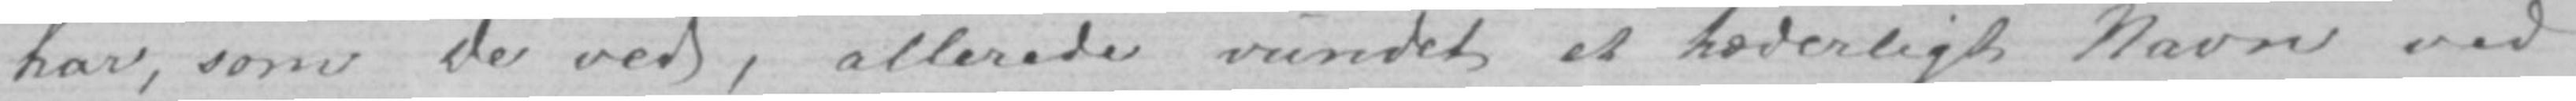har, som De ved, allerede vundet et hæderligt Navn ved
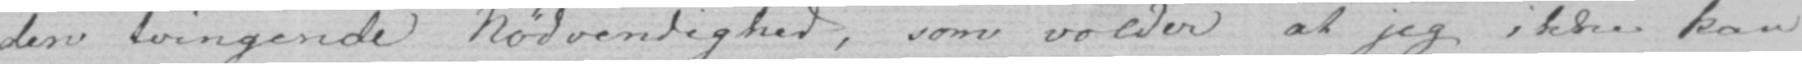den tvingende Nødvendighed, som volder at jeg ikke kan
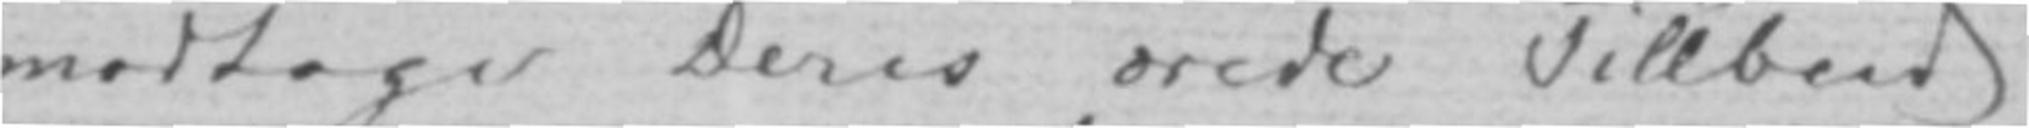modtage Deres ærede Tillbud.
